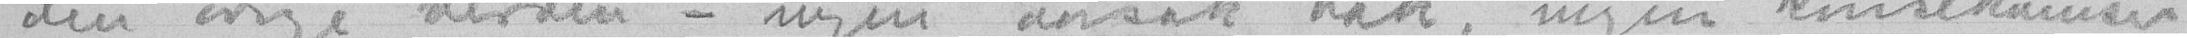den øvrige verden - ingen aarsak bak, ingen konsekvenser
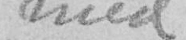med
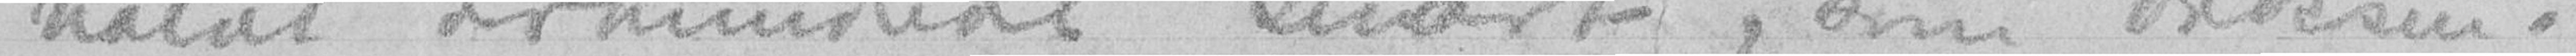halvt aahundrede snart, som voksen.
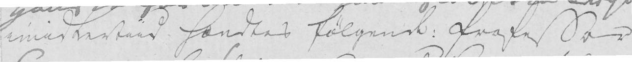imidtertiid hændtes følgende: Præste Søn
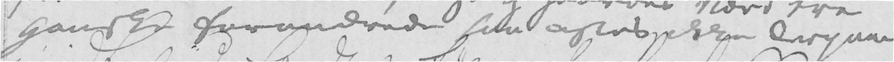ganske forandrede som opres ikke derpaa
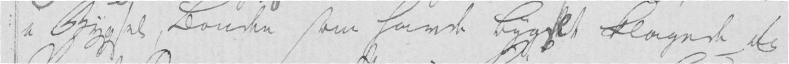i Bygsel, Bonden som havde bygsel klagede og
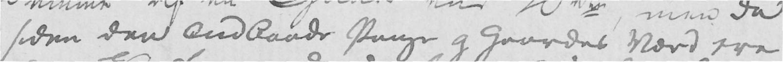siden den indbaade Penge og Gaardes Værd ere
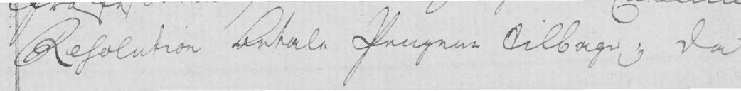Resolution betale Pengene tilbage; da
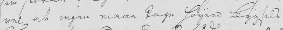vel at ingen maae tage høyere Bygsels
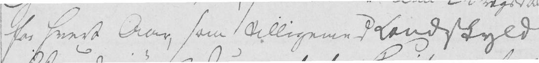for hvert Aar, som tilligemed Landskyld
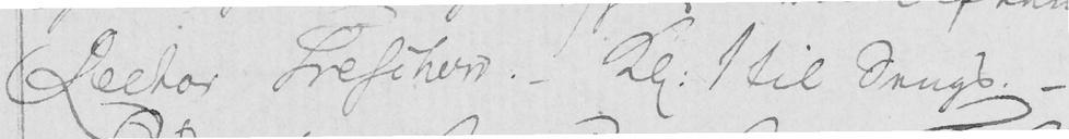Rector Treschow. - Kl. 1 til Sengs. -
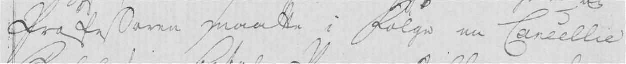Præste Sønen maatte i følge en Cancellie
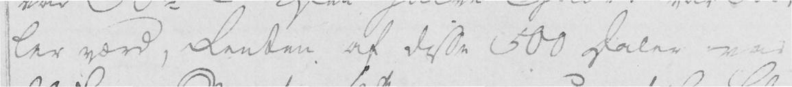ler værd, Renten af disse 500 Daler værd
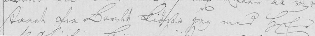staaet fra Bordet kiørte jeg med Hr
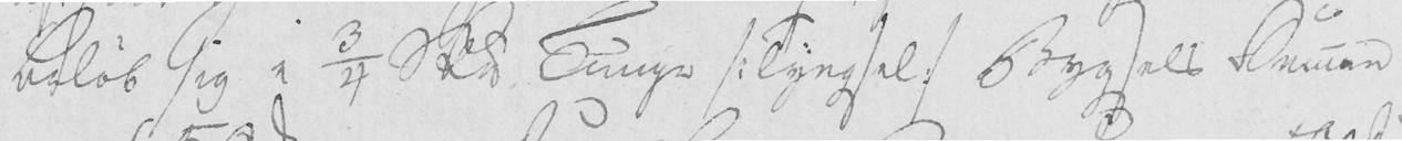beløb sig i 3/4 Skd Tings /: Tyngsel :/ Bygsels Formuen
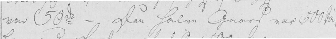var 50 rde - Den halve Gaard var 500 Da-
